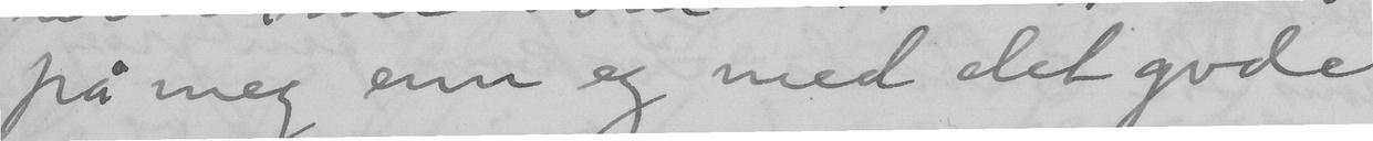på meg enn eg med det gode
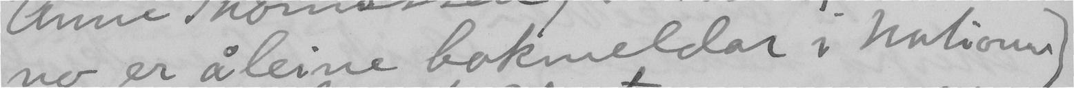no er åleine bokmeldar i Nationen,
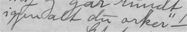igjen alt du orker" -
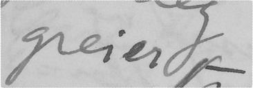greier –
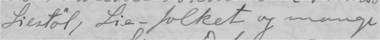Liestöl, Lie-folket og mange
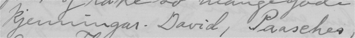kjenningar. David, Paasches,
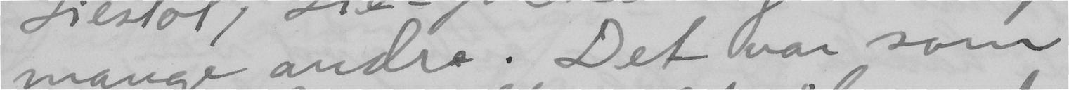mange andre. Det var som
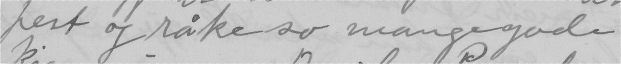fest og råke so mangegode
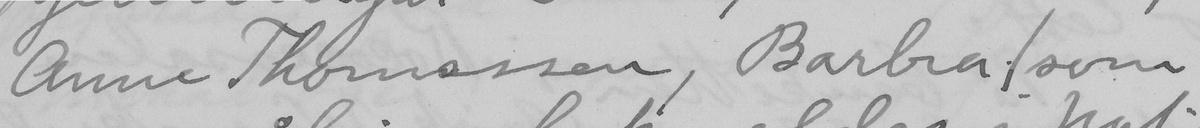Anne Thomassen, Barbra, som
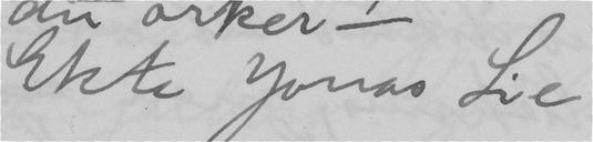Ekte Jonas Lie.
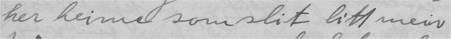her heime som slit litt meir
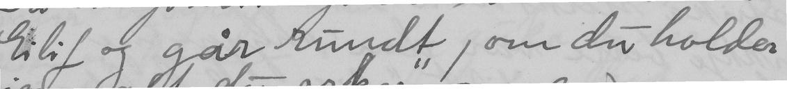Elif og går rundt, om du holder
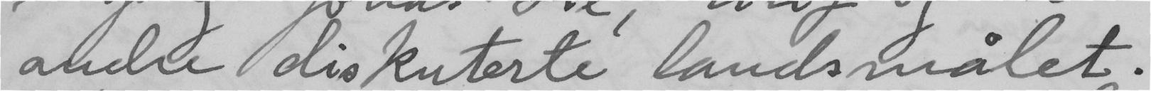andre diskuterte landsmålet.
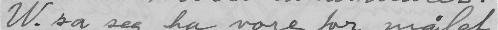W. sa seg ha vore for målet,
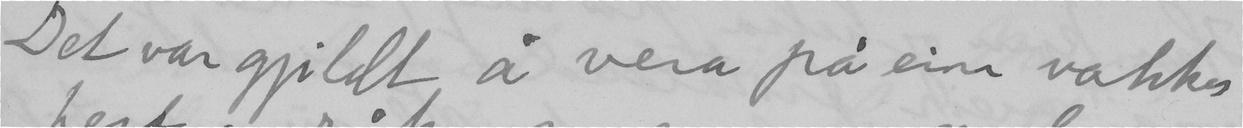Det var gjildt å vera på ein vakker
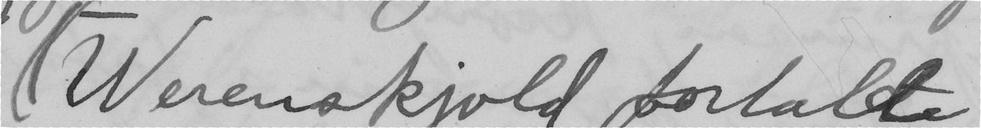Werenskjold fortalte
